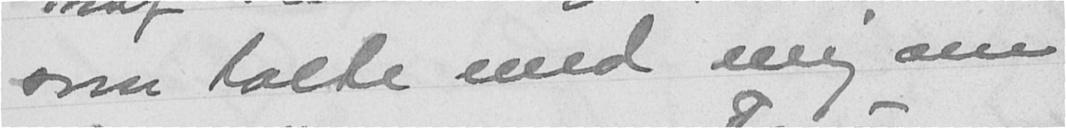som talte med mig om
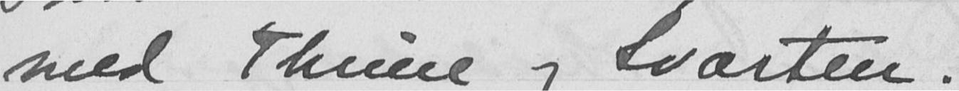med Thrine og Svarten.
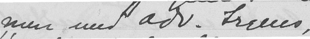men med adv. Irgens,
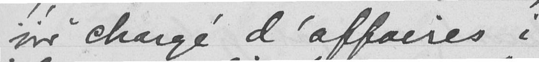"vor" chargé d'affaires i
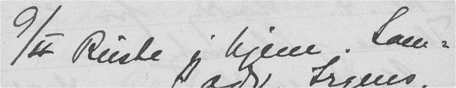9/II Reiste jeg hjem. Sam-
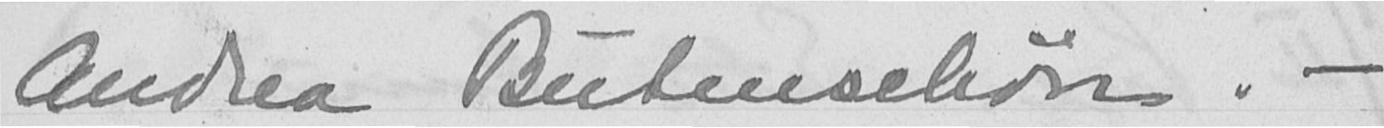Andrea Butenschøn. -
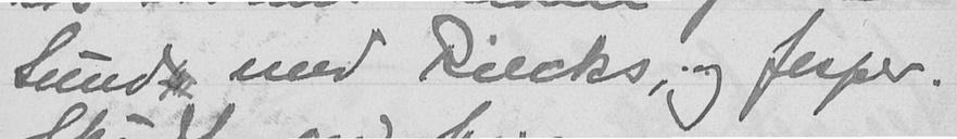Sund med Riecks, og Jesper.
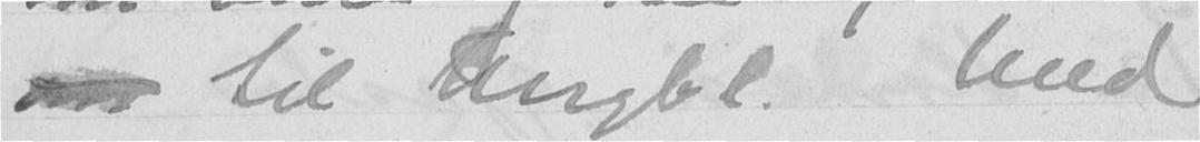om til Mrgbl. Med
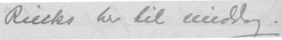Riecks her til middag.
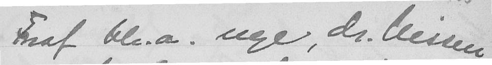Traf bl.a. nye, dr. Nissen
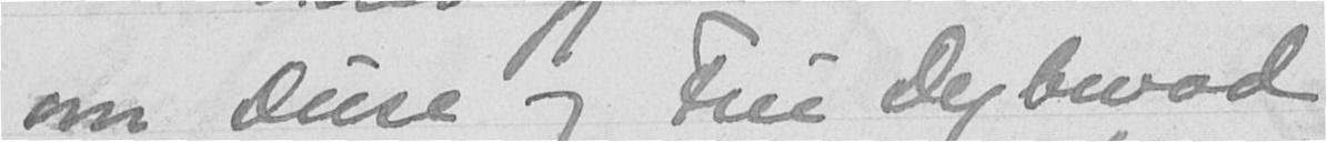om Duse og Fru Dybwad
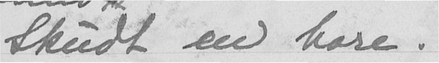Skudt en hare.
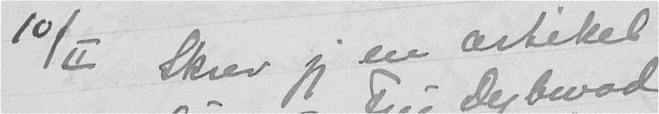10/II Skrev jeg en artikel
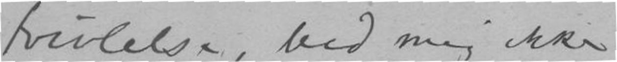tvivlelse, bed mig ikke
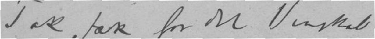Tak, tak for det Venskab,
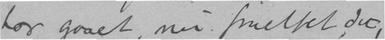har gaaet, nu smeltet du,
